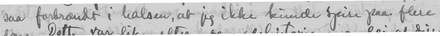saa forbrændt i halsen, at jeg ikke kunde spise paa flere
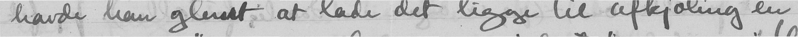havde han glemt, at lade det ligge til afkjøling en
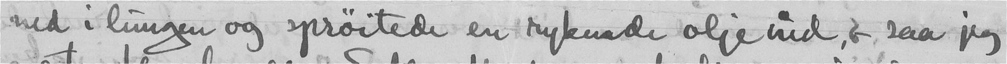ned i lungen og sprøitede en sykende olje ind, - saa jeg
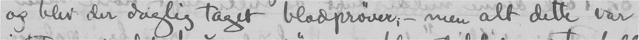og blev der daglig taget blodprøver, - men alt dette var
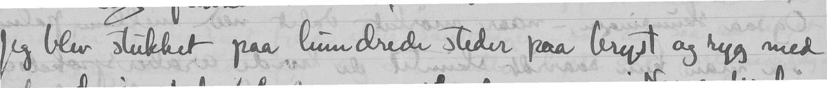Jeg blev stukket paa hundrede steder paa bryst og ryg med
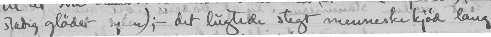stadig glødet sylen); - det lugtede stegt menneskekjød lang
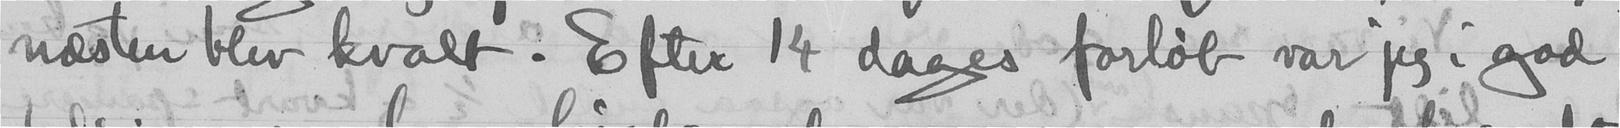næsten blev kvalt. Efter 14 dages forløb var jeg i god
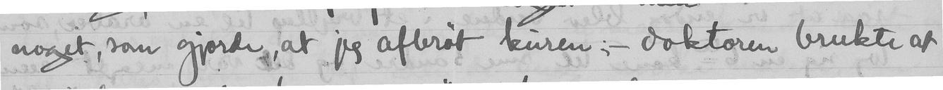noget, som gjorde, at jeg afbrøt kuren ; - doktoren brukte at
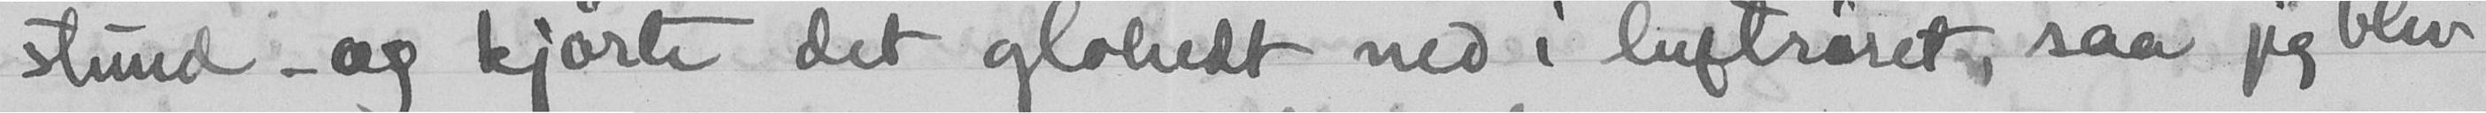stund - og kjørte det glohedt ned i luftrøret, saa jeg blev
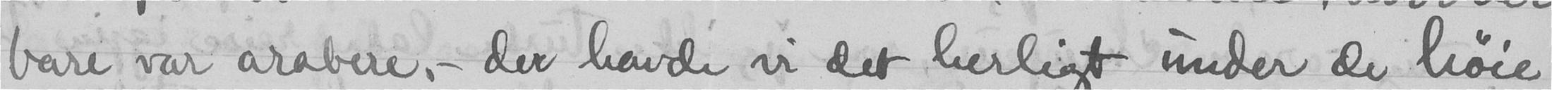bare var arabere, - der havde vi det herligt under de høie
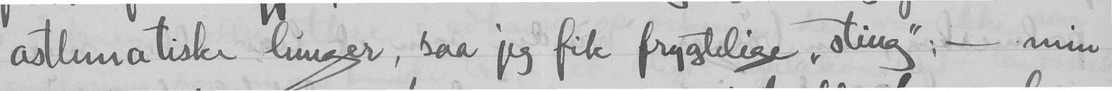asthmatiske lunger, saa jeg fik frygtelige "sting" - min
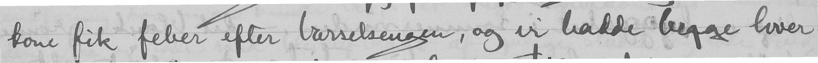kone fik feber efter barselsengen, og vi hadde begge hver
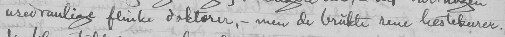usedvanlige flinke doktorer, - men de brukte rene hestekurer.
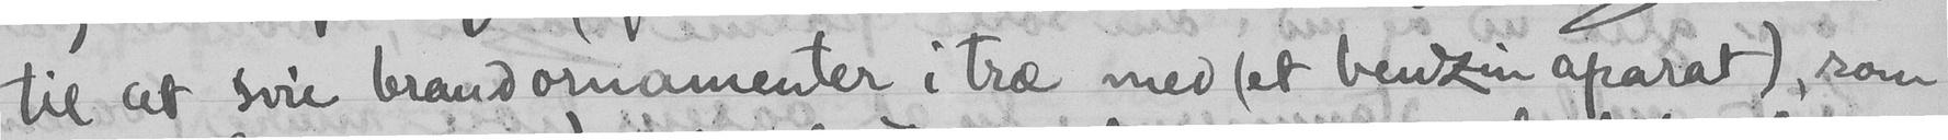til at svie brandornamenter i træ med (et benzin aparat), som
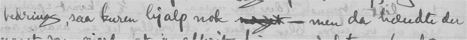bedring, saa kuren hjalp nok  - men da hændte der
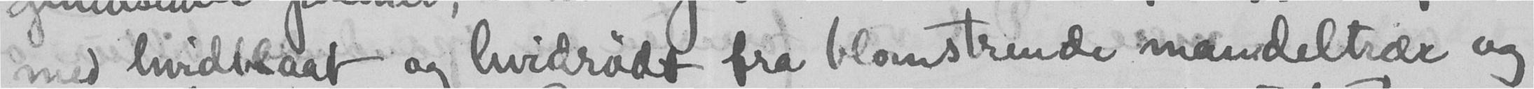med hvidblaat og hvidrødt fra blomstrende mandeltrær og
